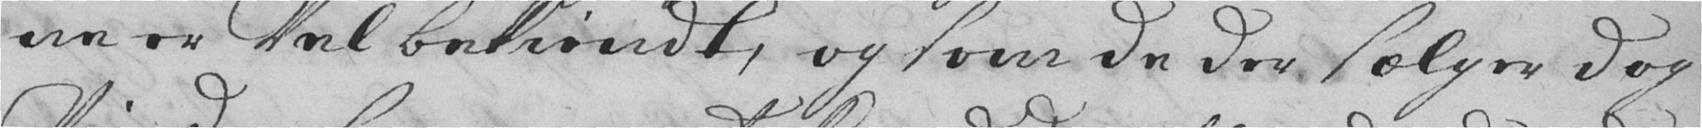ne er Vel bekiendt, og som de der sælger dog
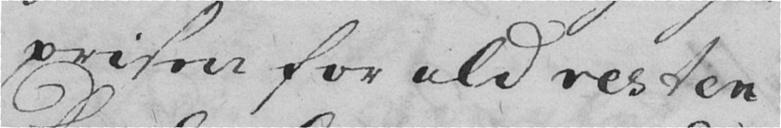prisen for ald resten
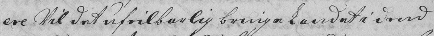ere vil det ufeilbarlig bringe Landet i dend
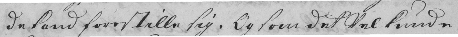de kand forestille sig. Og som det Vel kunde
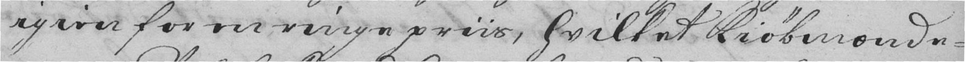igien for en ringe priis, hvilket Kiøbmænde-
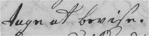tage at bevise.
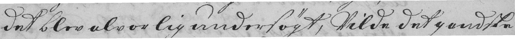det blev alvorlig undersøgt, vilde det gandske
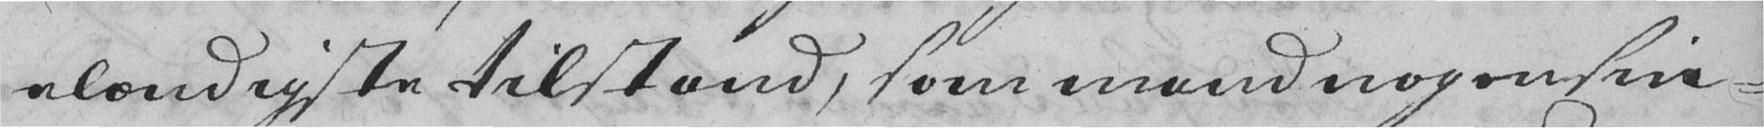elændigste tilstand, som mand nogen sin-
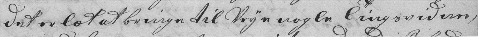det er læt at bringe til Veye nogle Tingsvidner,
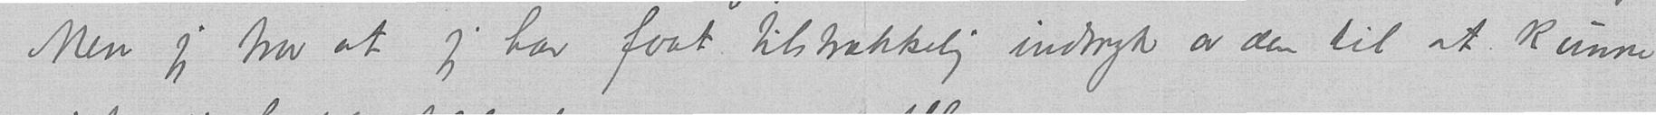Men jeg tror at jeg har faat tilstrækkelig indtryk av den til at kunne
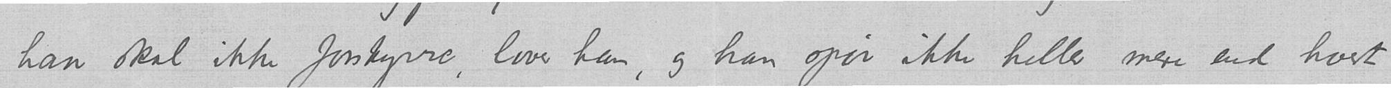han skal ikke forstyrre, lover han, og han spør ikke heller mere end hvert
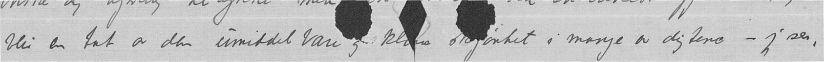blir en tat av den umiddelbare og klare skjønhet i mange av digtene – jeg ser,
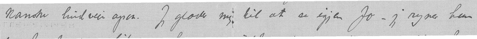kanske lindveir ogsaa. Jeg glæder mig til at se igjen Jo – jeg regner ham
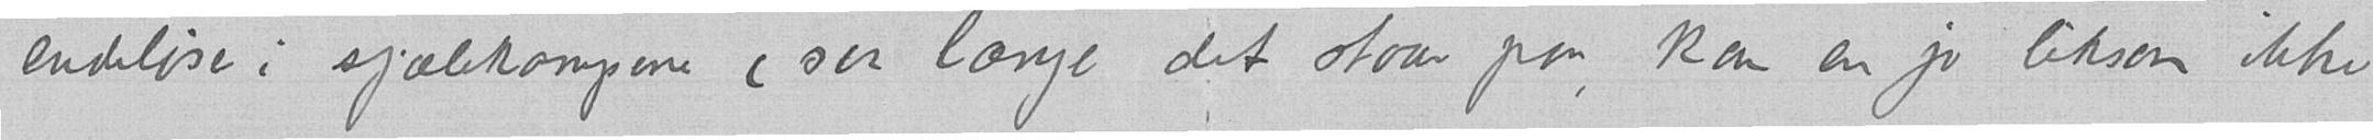endeløse i sjælekampene (saa længe det staar paa, kan en jo liksom ikke
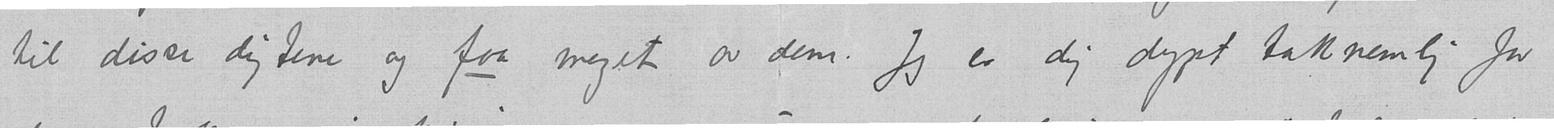til disse digtene og faa meget av dem. Jeg er dig dypt taknemlig for
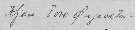Kjære Tore Ørjasæter.
